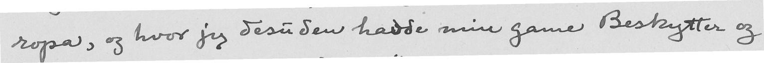ropa, og hvor jeg desuden hadde min game Beskytter og
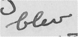blev
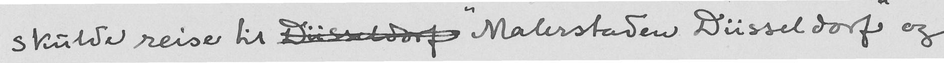skulde reise til Düsseldorf "Malerstaden Düsseldorf" og
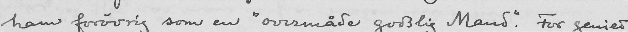ham forøvrig som en "overmåde godslig Mand". For geniet
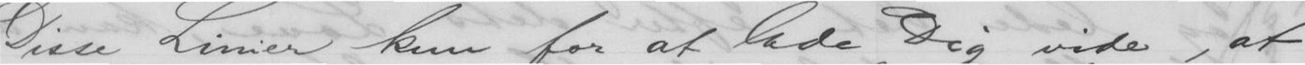Disse Linier kun for at lade Dig vide, at
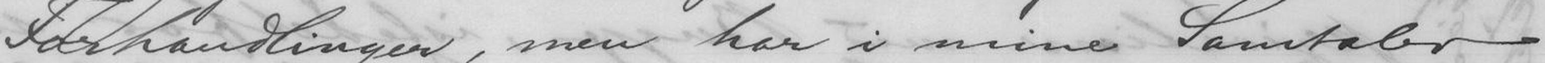Forhandlinger, men har i mine Samtaler
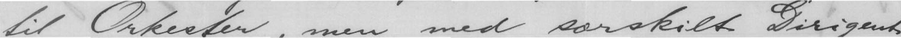til Orkester, men med særskilt Dirigent.
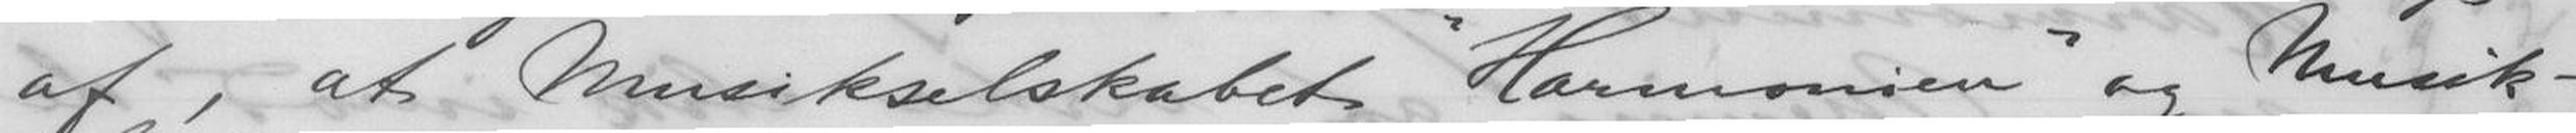af, at Musikselskabet "Harmonien" og Musik-
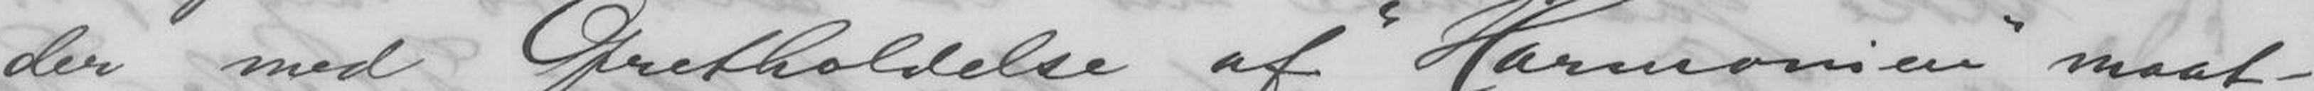der med Opretholdelse af "Harmonien" maat-
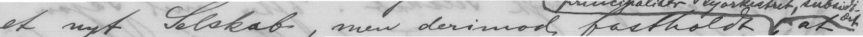et nyt Selskab, men deimod fastholdt at
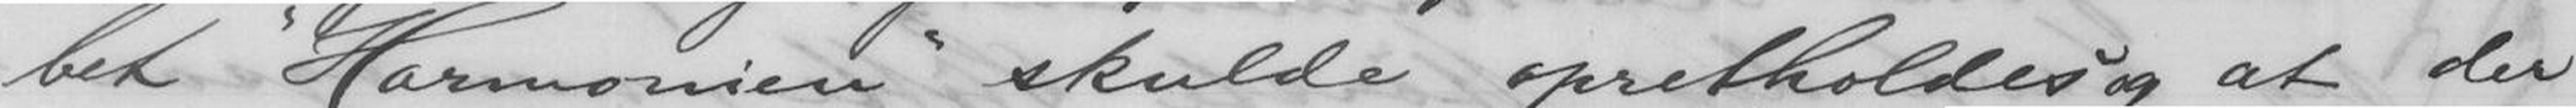bet "Harmonien" skulde opretholdes og at der
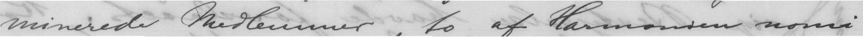minerede Medlemmer, to af Harmonien nomi-
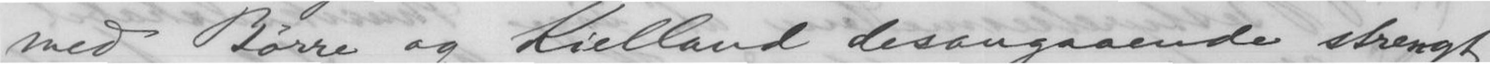med Børre og Kielland desangaaende strengt
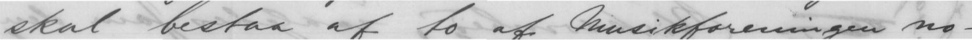skal bestaa af to af Musikforeningen no-
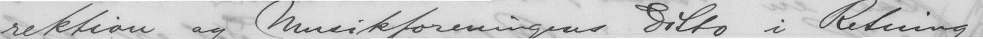rektion og Musikforeningens Ditto i Retning
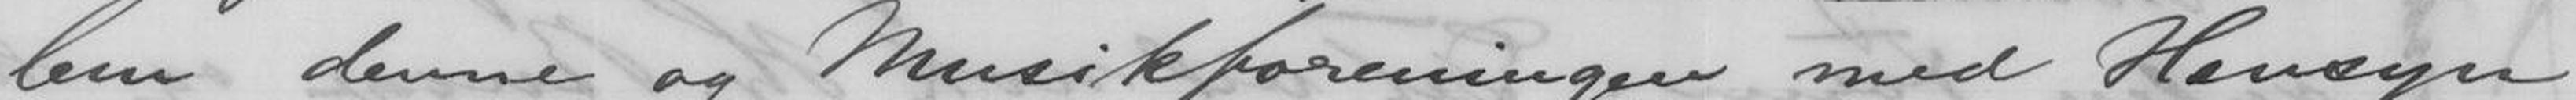lem denne og Musikforeningen med Hensyn
